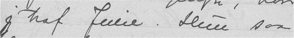jeg traf Julie. Hun saa
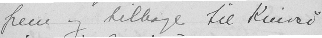frem og tilbage til Knivsø
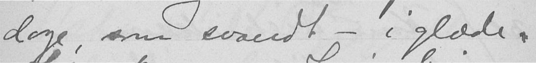dage, som svandt - i glæde.
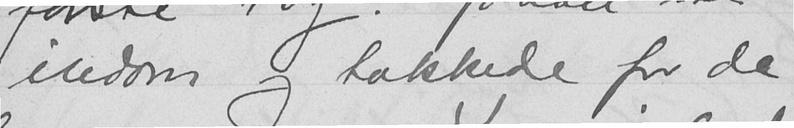indom og takkede for de
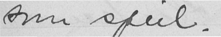som speil.
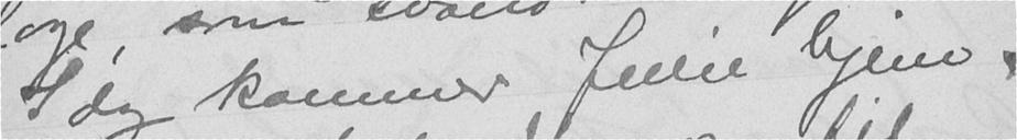I dag kommer Julie hjem,
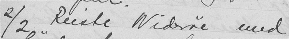2/2 Reiste Widerøe med
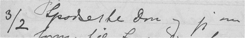3/2 Spadserte Don og jeg om
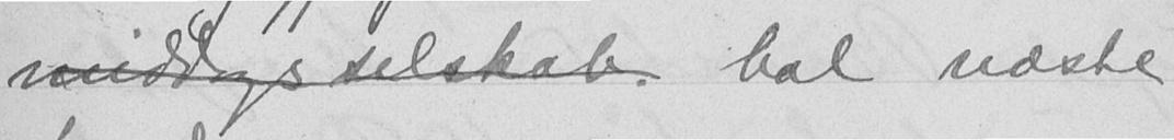middagsselskab. bal næste
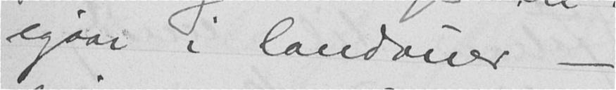igaar i landveier -
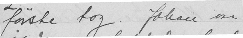første tog. Johan var
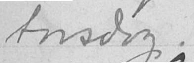torsdag.
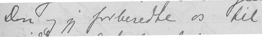Don og jeg forberedte os til
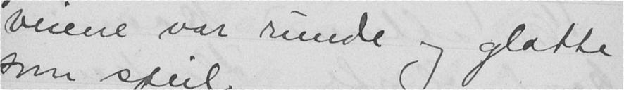veiene var runde og glatte
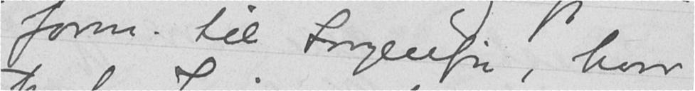form. til Sorgenfri, hvor
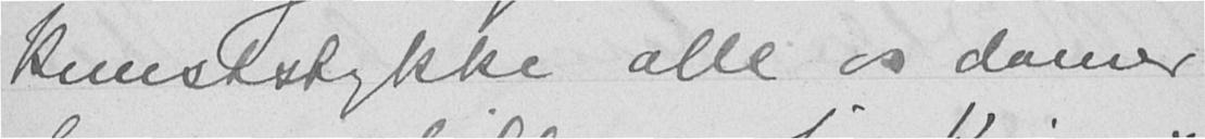kunststykke alle os damer
